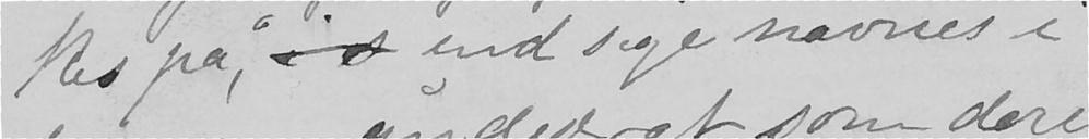kes på, end sige nævnes i
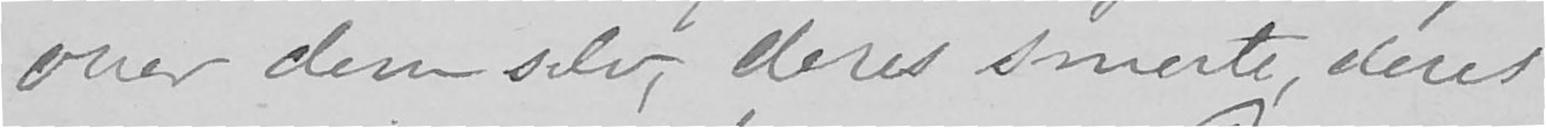over dem selv, deres smerte, deres
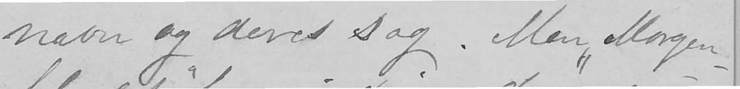navn og deres sag. Men "Morgen-
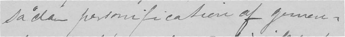sådan personification af gemen-
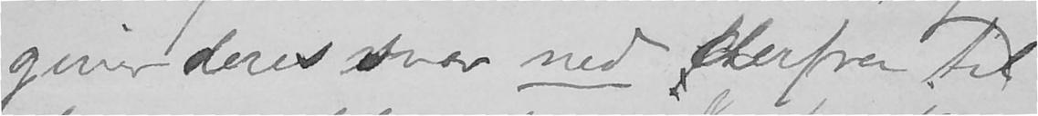giver deres svar ned derfra til
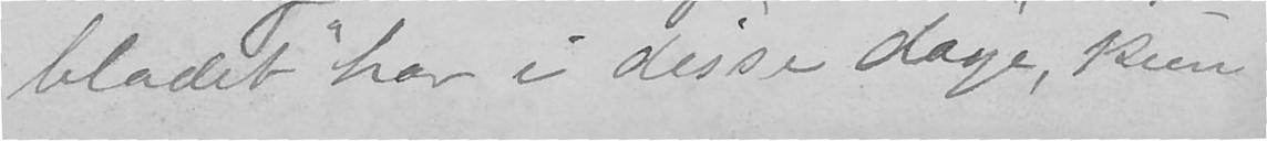bladet" har i disse dage, kun
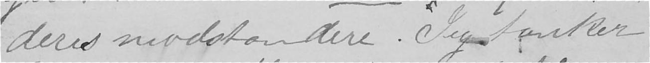deres modstandere. Jeg tænker
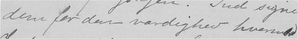dem for deres værdighed hvormed
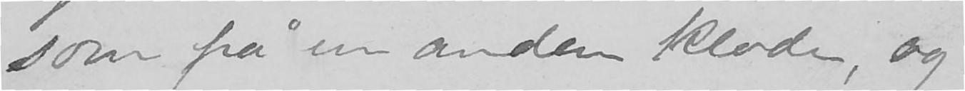som på en anden klode, og
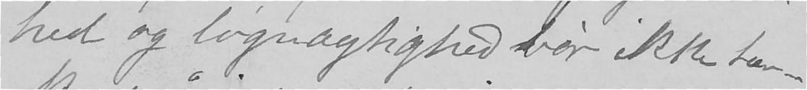hed og løgnagtighed bør ikke tæn-
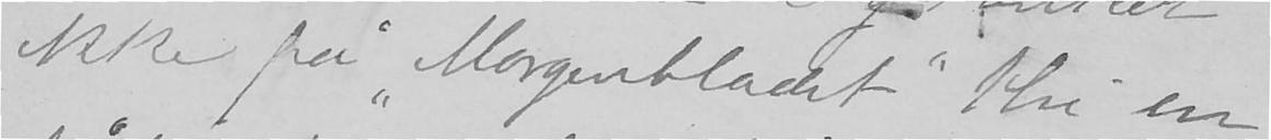ikke på "Morgenbladet" thi en
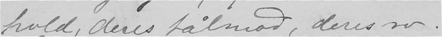hold, deres tålmod, deres ro.
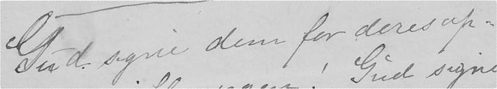Gud signe dem for deres op-
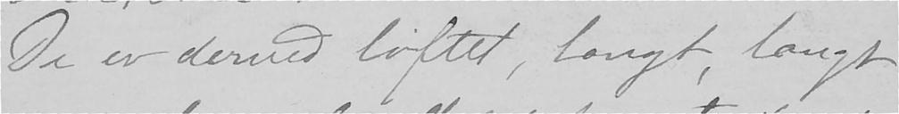De er dermed løftet, langt, langt
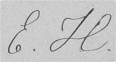E. H.
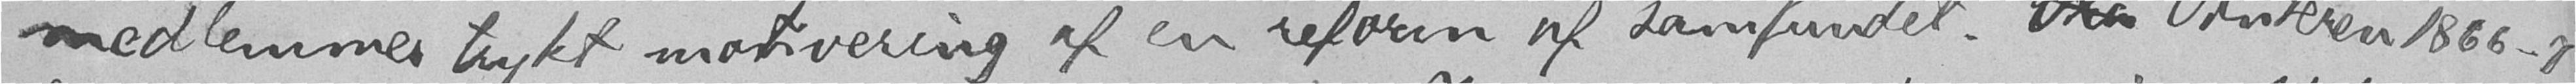medlemmer trykt motivering af en reform af samfundet.  Vinteren 1886-7
